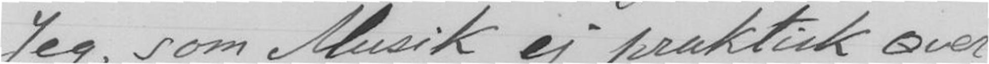Jeg, som Musik ej praktisk øver,
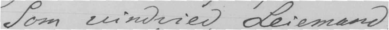Som vindvied Leimand,
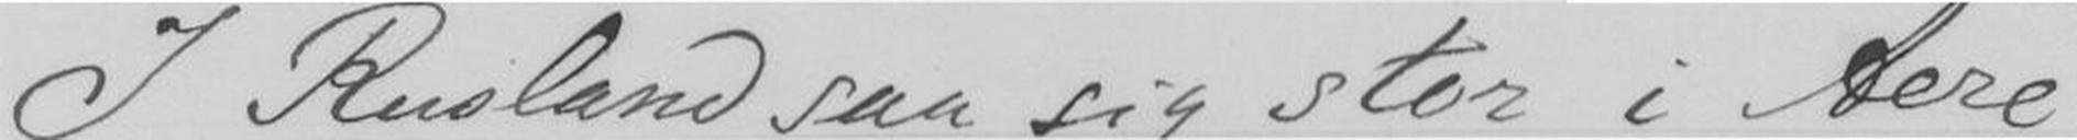I Rusland saa sig stor i Aere
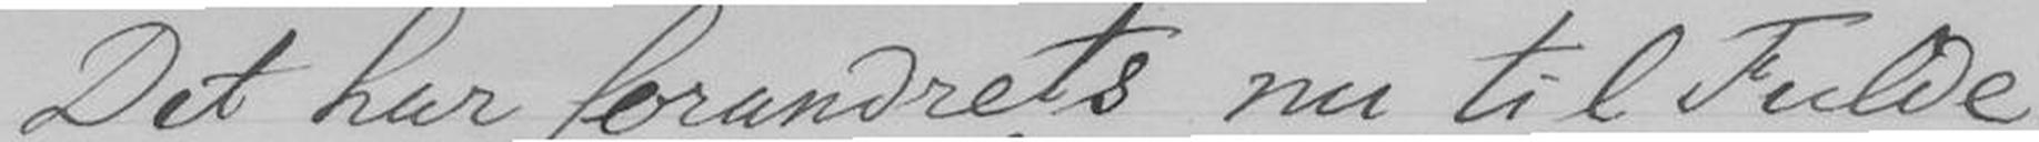Det har forundrets nu til Fulde
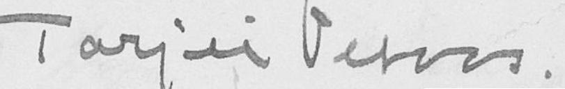Tarjei Vesaas
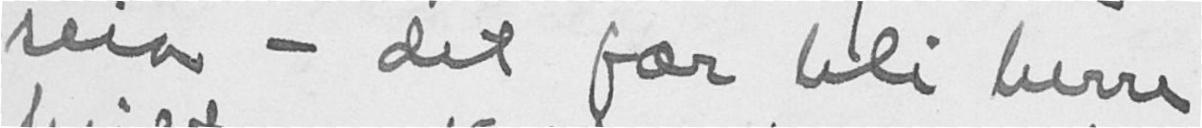seia - det fær liti herre
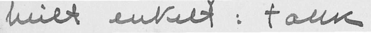hult enkelt: Takk!
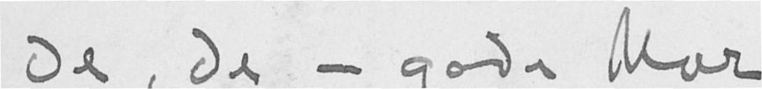Ja, Ja – goda Mor
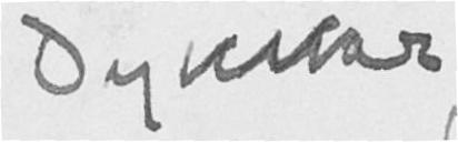Dykkar
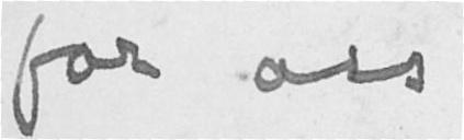for oss.
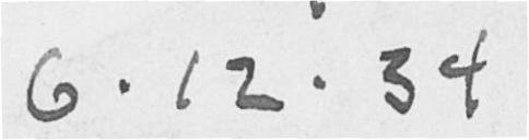6.12.34
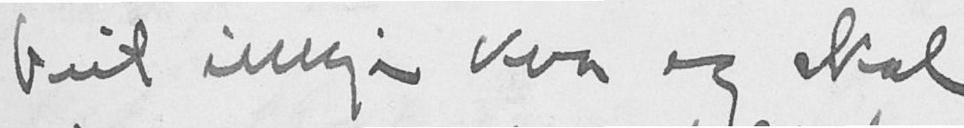Veit ikje kva eg skal
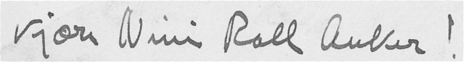Kjære Nini Roll Anker.
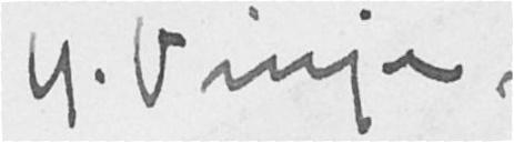G. Vinja,
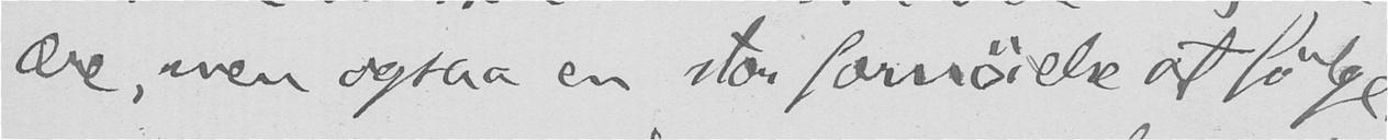ære, men ogsaa en stor fornøielse at følge.
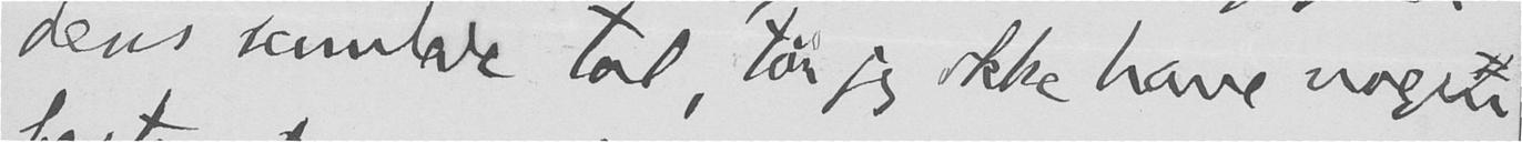dens samlede tal, tør jeg ikke have nogen
